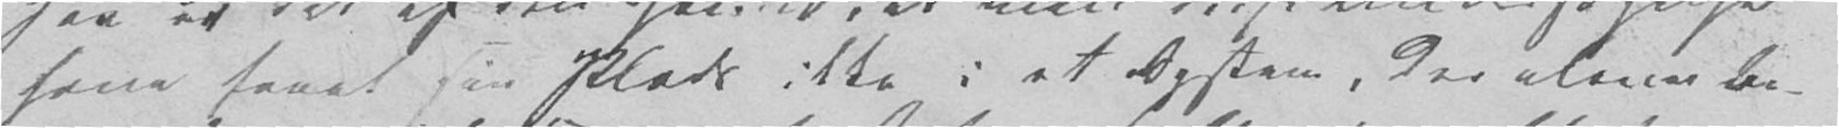have faaet sin Plads ikke i et System, der alene be-
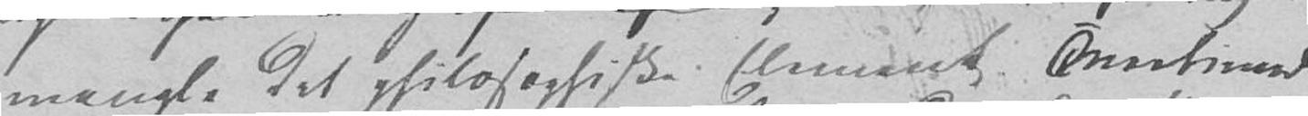mangle det philosophiske Element. Tvertimod
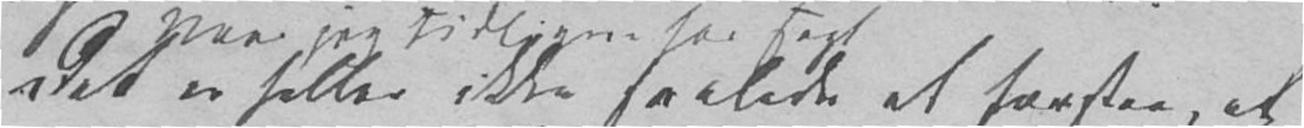Det er heller ikke saaledes at forstaa, at
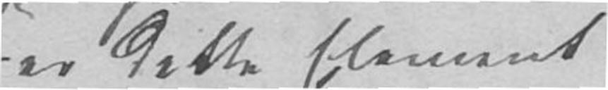er dette Element
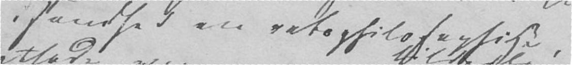isandhed en retsphilosophisk,
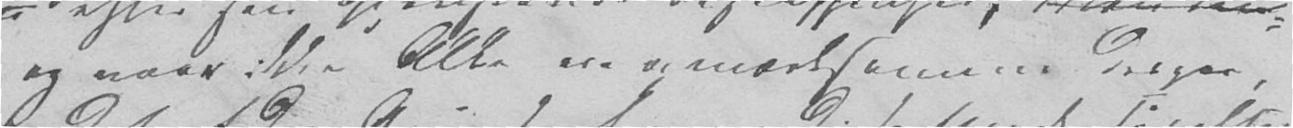og naar ikke Alle ere opmærksomme derpaa,
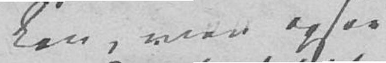Lov, men ogsaa
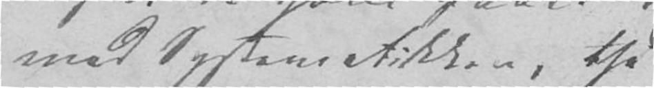med Systematikken, thi
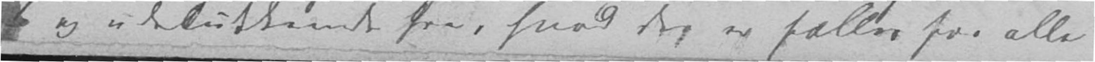og udelukkende fra, hvad der er fælles for alle
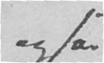ogsaa
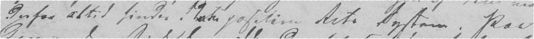derfor altid findes i den positive Rets System. Paa
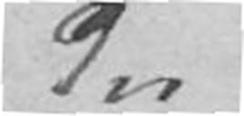der
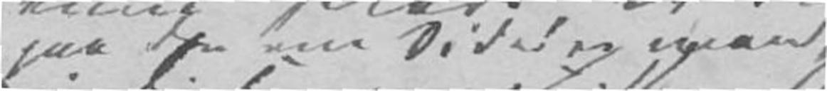paa den ene Side er man
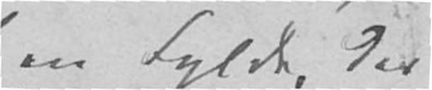en Fylde, der
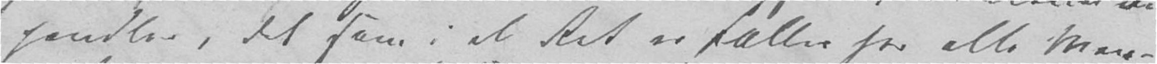handler, det som i al Ret er fælles for alle Men-
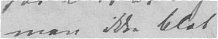man ikke blot
